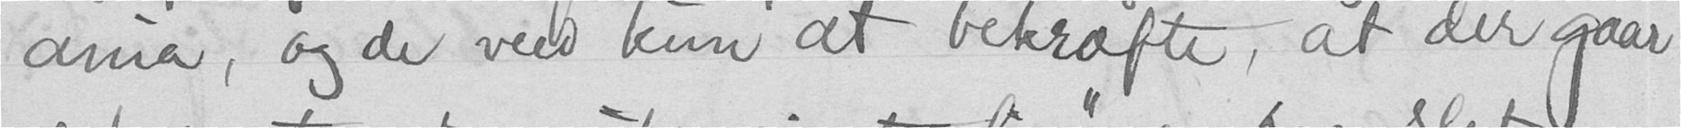ania, og de veed kun at bekræfte, at der gaar
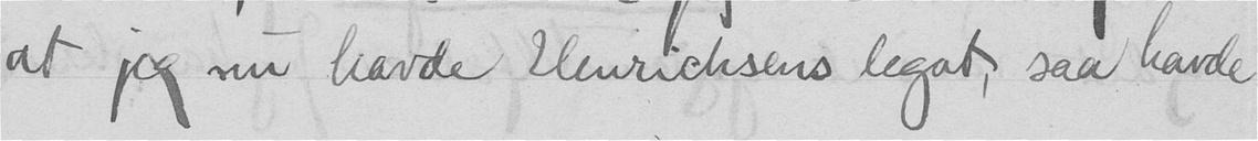at jeg nu havde Henrichsens leget, saa havde
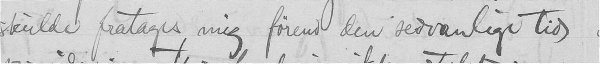skulde fratages mig førend den sedvanlige tid
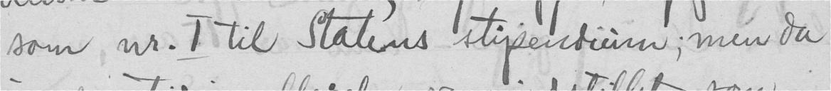som nr.1 til statens stipendium; men da
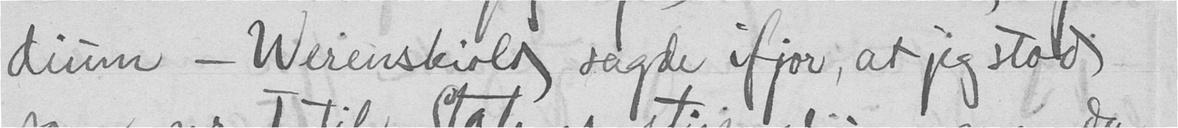dium - Werenskiold sagde ifjor, at jeg stod
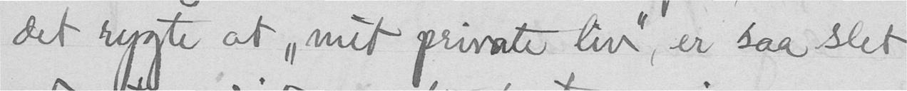det rygte at "mit private liv, er saa slet
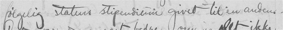følgelig statens stipendiun givet til en anden.
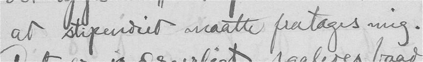at stipendet maatte fratages mig.
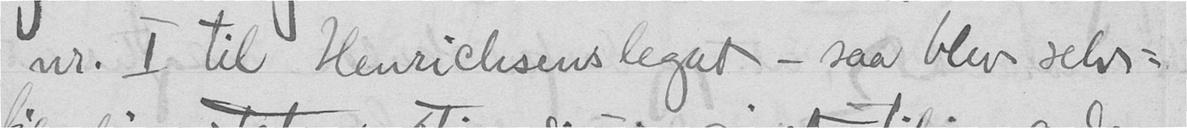nr.1 til Henrichsens leget, - saa blev selv-
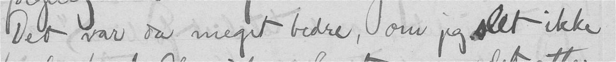Det var da meget bedre, om jeg slet ikke
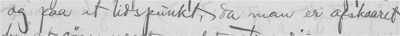og paa et tidspunkt, da man er afskaaret
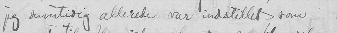jeg samtidig allerede var indstillet som
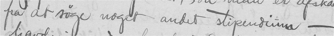fra at søge noget andet stipendium -
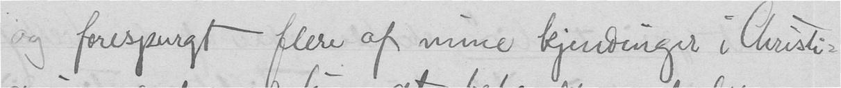og forespurgt flere af mine kjendinger i Christi-
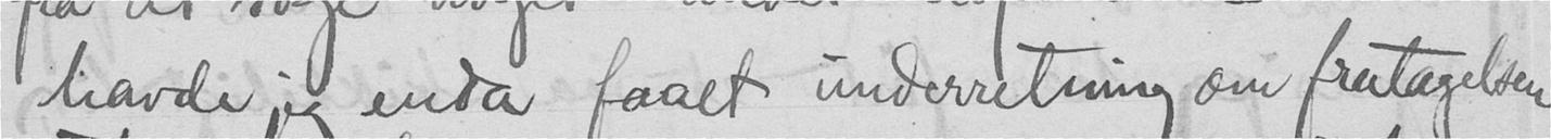havde jeg enda faaet underretning om fratagelsen
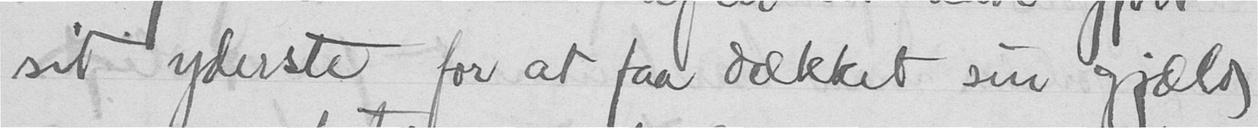sit yderste for at faa dækket sin gjæld
